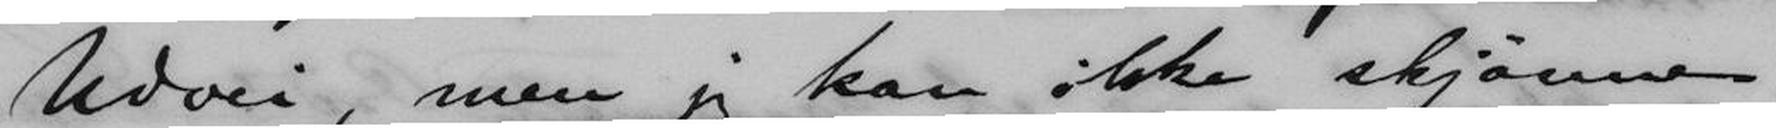Udvei, men jeg kan ikke skjønne
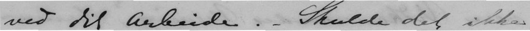ved dit Arbeide. - Skulde det ikke
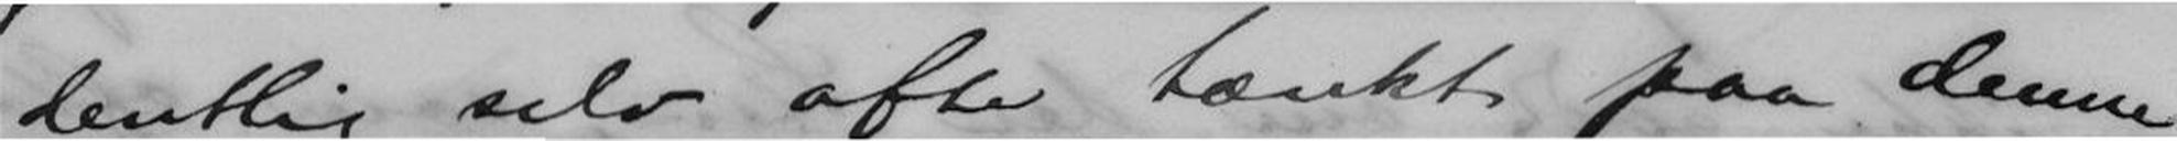dentlig selv ofte tænkt paa denne
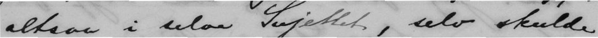altsaa i selve Sujettet, selv skulde
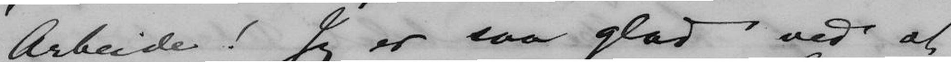Ardeide! Jeg er saa glad ved at
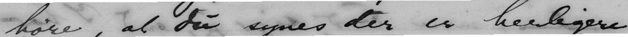høre, at de synes der er herligere
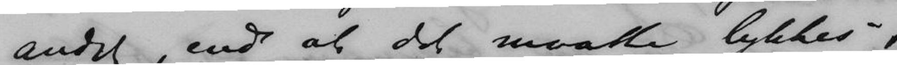andet, end at det maatte lykkes,
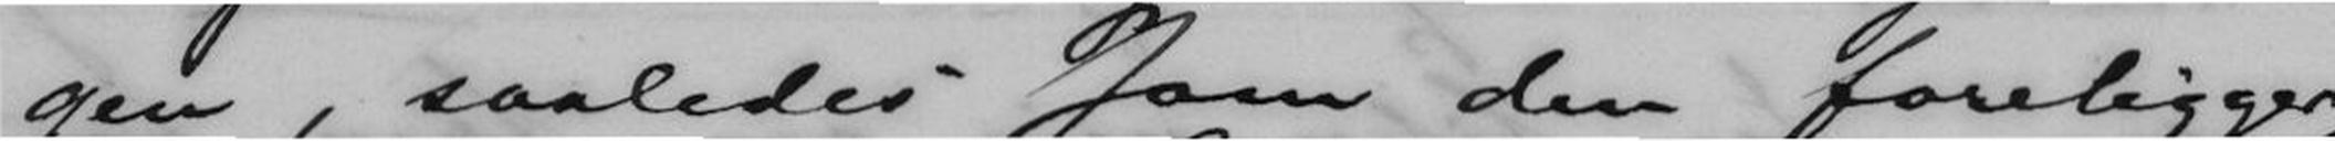gen, saaledes som den foreligger,
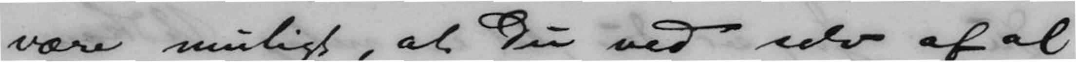være muligt, at Du ved selv af al
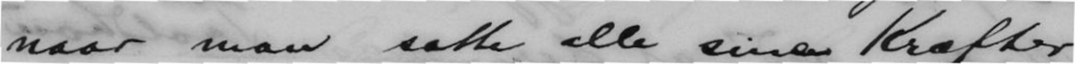naar man satte alle sine Kræfter
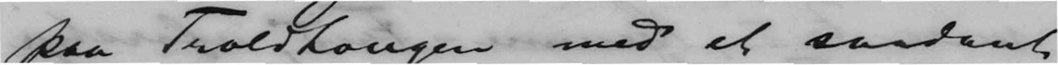paa Troldhougen med et saadant
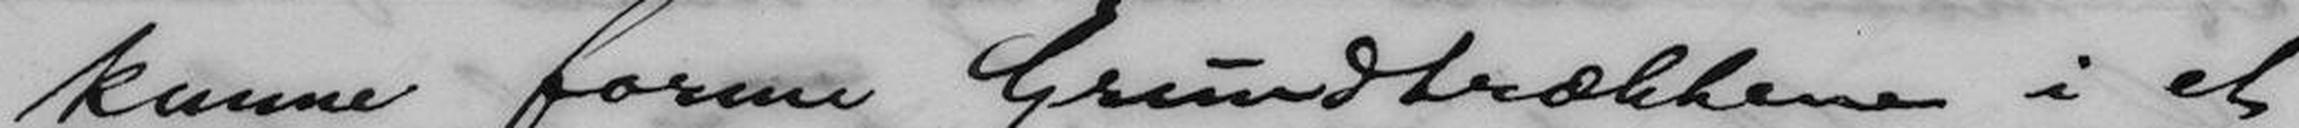kunne forme Grundtrækkene i et
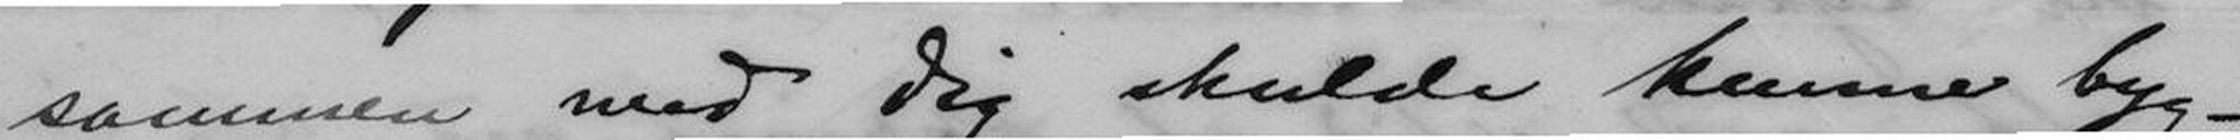sammen med Dig skulde kunne byg-
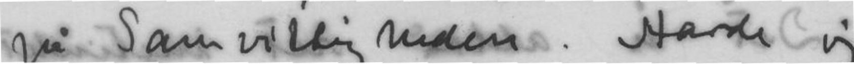på Samvittigheden. Havde jeg
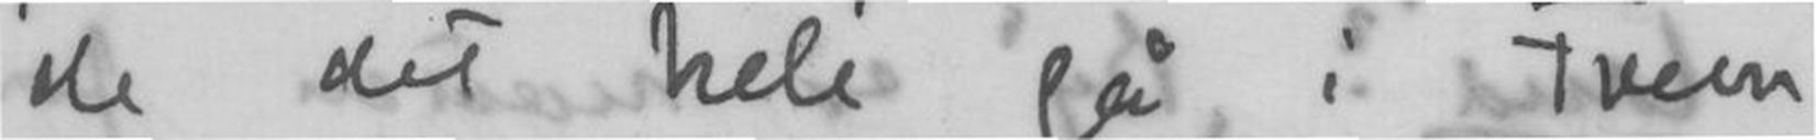de det hele gå i Frem
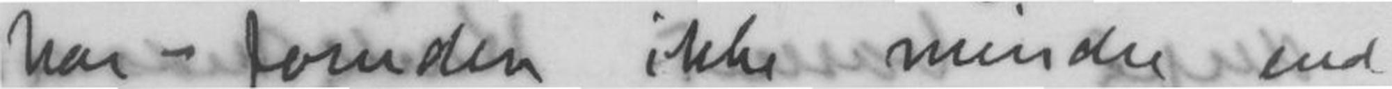har - foruden ikke mindre end
